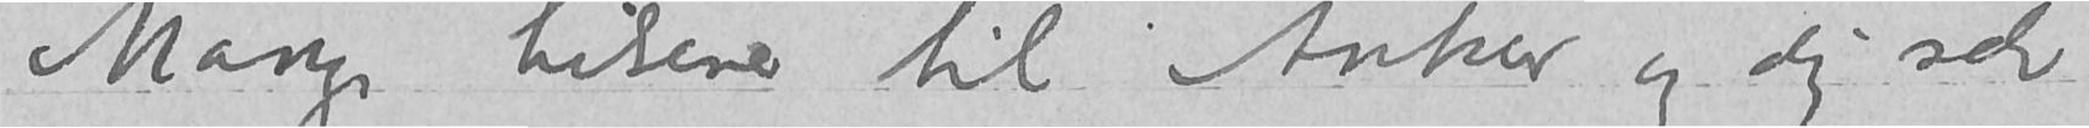Mange hilsener til Anker og dig selv
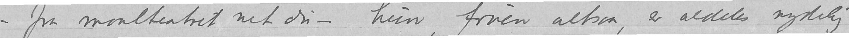fra maalteatret vet du – hun, fruen altsaa, er aldeles nydelig
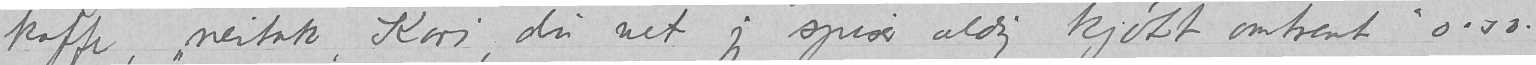kaffe, «neitakk, Kari, du vet jeg spiser aldrig kjøtt omtrent» o.s.v. –
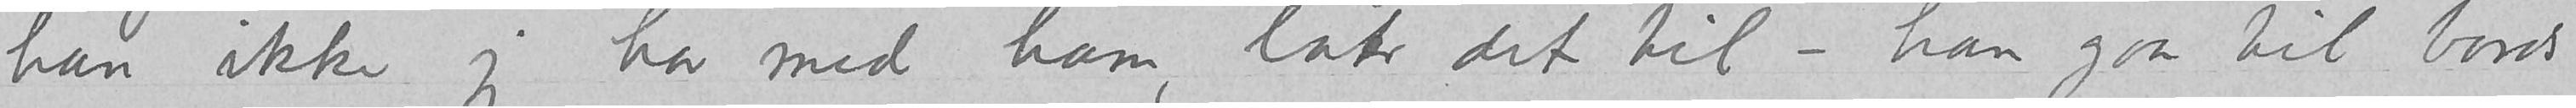han ikke jeg har med ham, later det til – han gaar til bords
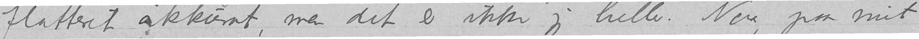flatteret akkurat, men det er ikke jeg heller. Naa, paa mit
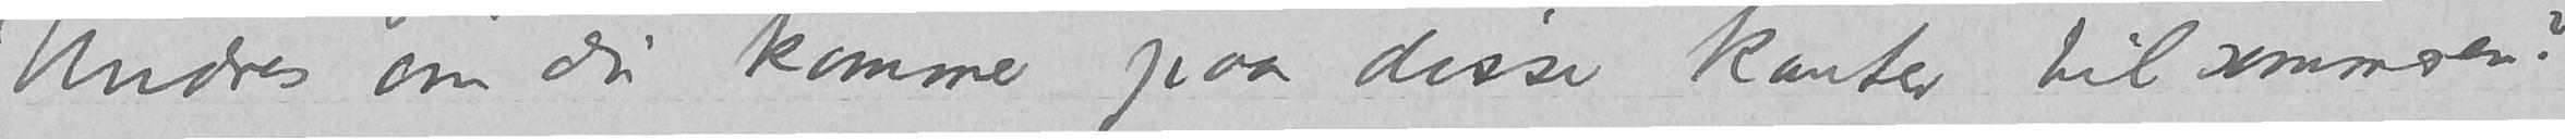Undres om du kommer paa disse kanter til sommeren?
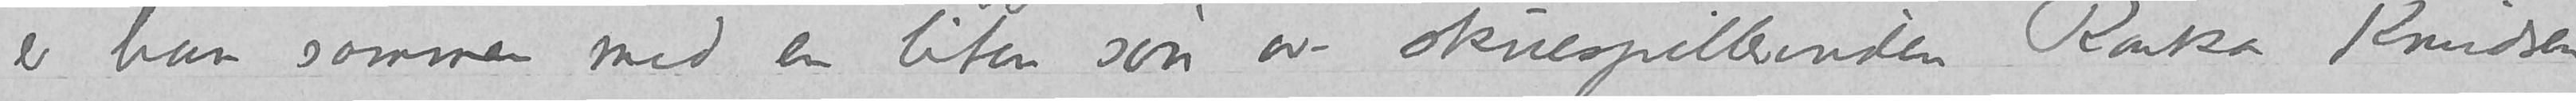er han sammen med en liten søn av skuespillerinden Ranka Knudsen
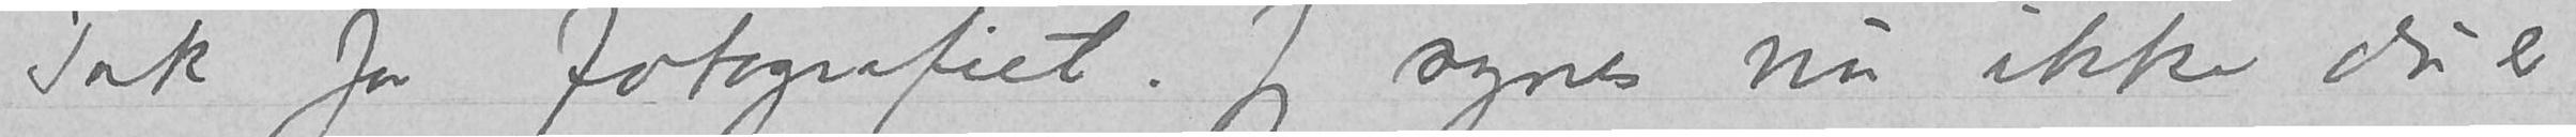Tak for fotografiet. Jeg synes nu ikke du er
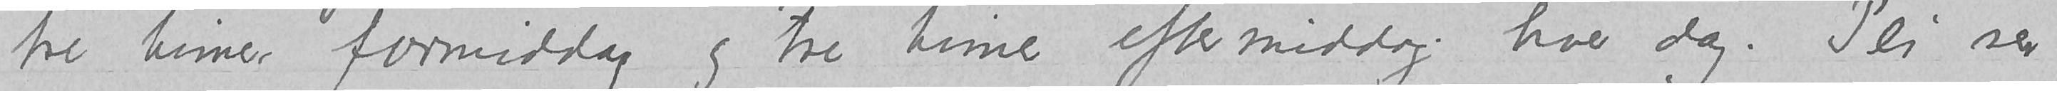tre timer formiddag og tre timer eftermiddag hver dag. Pei ser
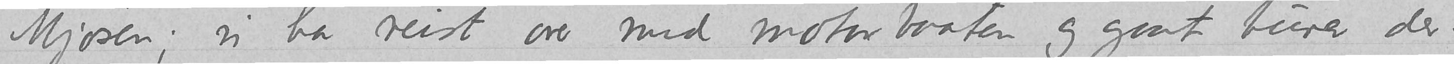Mjøsen; vi har reist over med motorbaaten og gaat turer der.
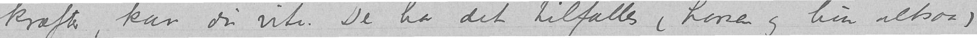kræfter, kan du vite. De har det tilfælles (Larsen og hun altsaa)
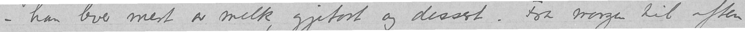han lever mest av melk, gjetost og dessert. Fra morgen til aften
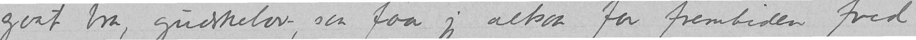gaat bra, gudskelov, saa faar jeg altsaa for fremtiden fred
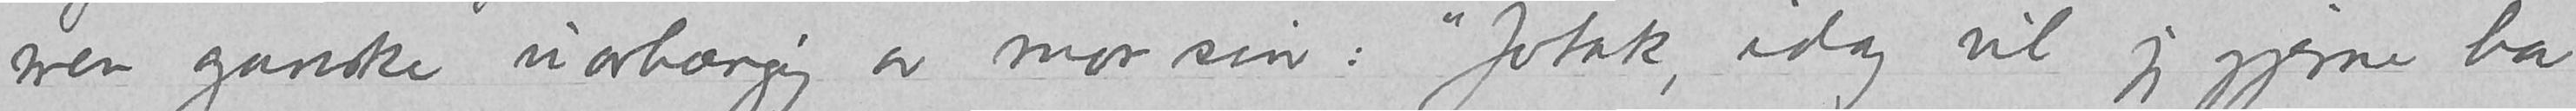men ganske uavhængig av mor sin: «Jotak, idag vil jeg gjerne ha
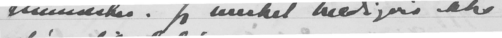mennesker. Jeg resket heldigvis ikke
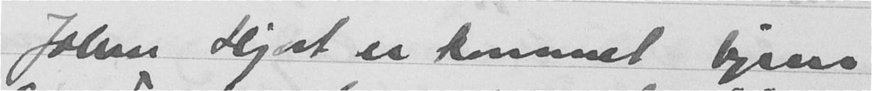Johan Hjort er kommet hjem
